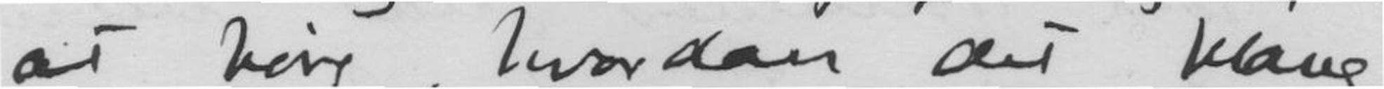at høre, hvordan det klang,
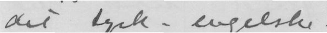det tysk - engelske.
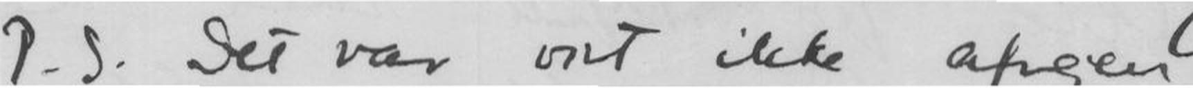P.S. Det var vist ikke afvejen,
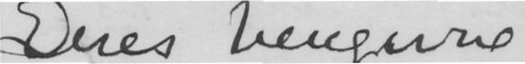Deres hengivne
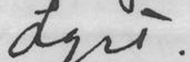Lyst.
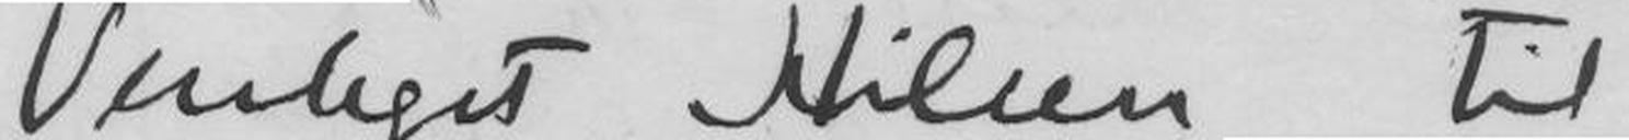Venligst Hilsen til
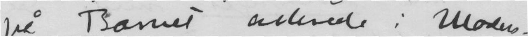på Barnet allerede i Moders-
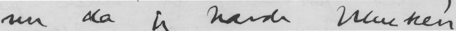nu da jeg havde Musiken
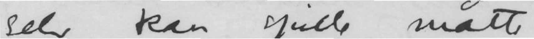selv kan spille måtte jeg
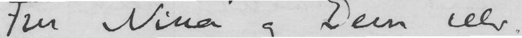Fru Nina og Dem selv.
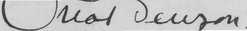Otto Benzon.
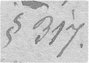§ 317.
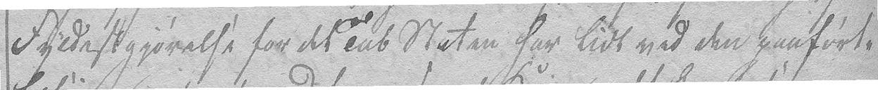Fyldestgjørelse for det Tab Staten har lidt ved den paaførte
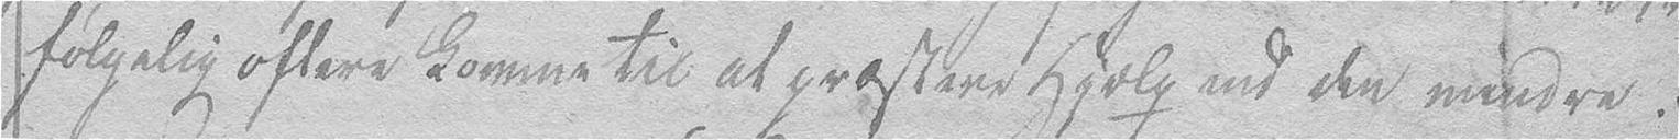følgelig oftere komme til at præstere Hjælp end den mindre.
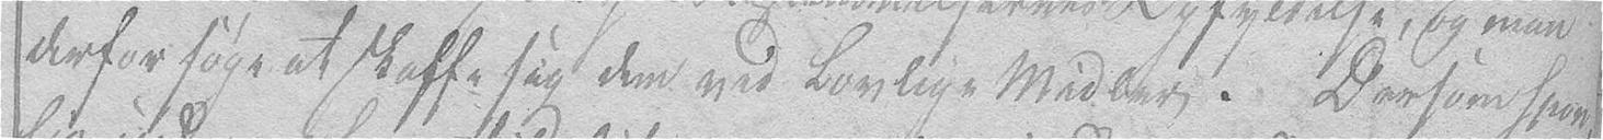derfor søge at skaffe sig dem ved Lovlige Midler. Dersom hene-
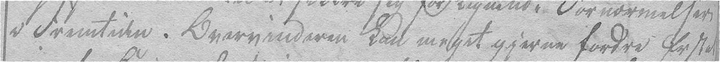i Fremtiden. Overvinderen kan meget gjerne fordre Erstat-
